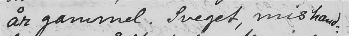år gammel. Sveget mishand-
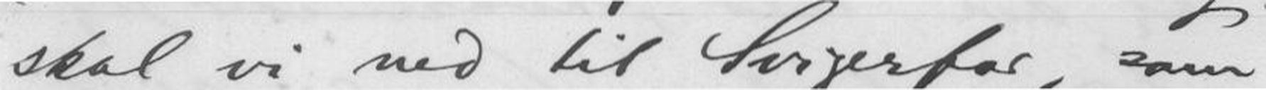skal vi ned til Svigerfar, som
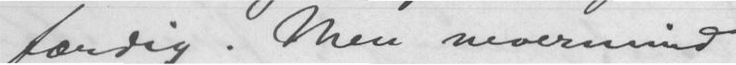færdig. Men nevermind,
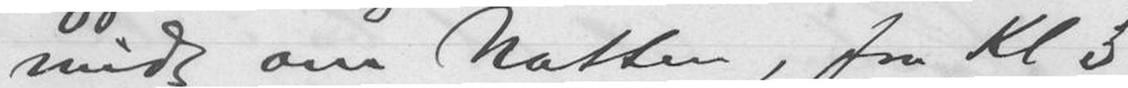midt om Natten, fra Kl 3
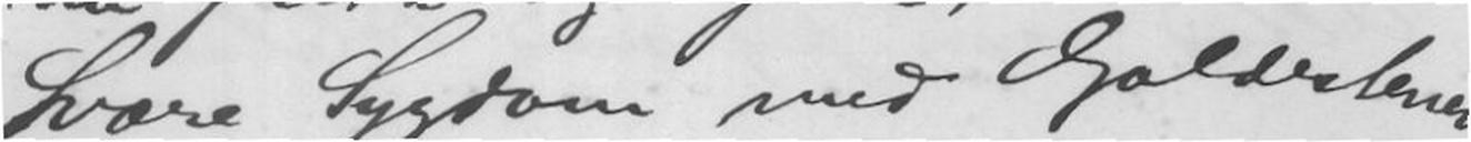svære Sygdom med Galdestenene
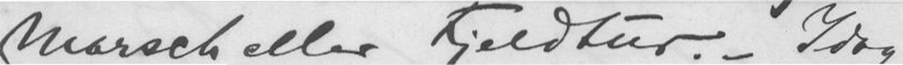Marsch eller Fjeldtur. - Idag
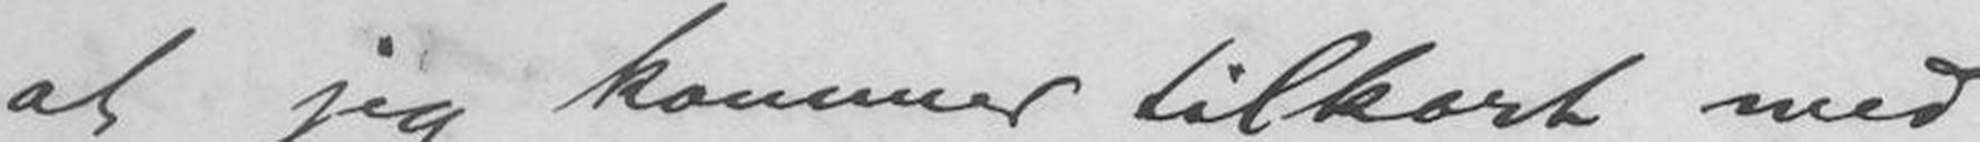at jeg kommer tilkort med
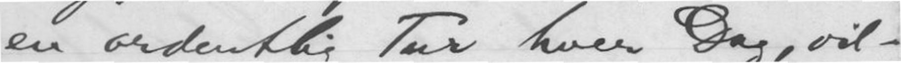en ordentlig Tur hver Dag, vil-
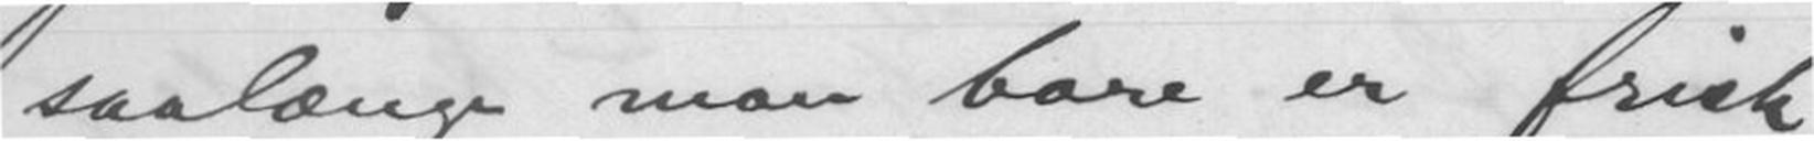saalænge man bare er frisk
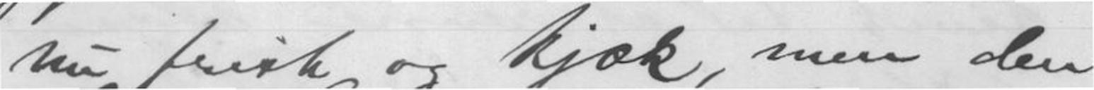nu frisk og kjæk, men den
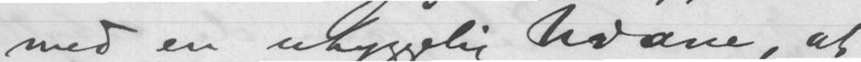med en uhyggelig Uvane, at
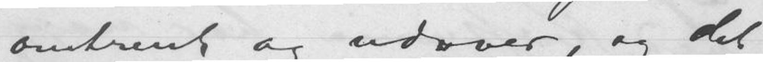omtrent og udover, og det
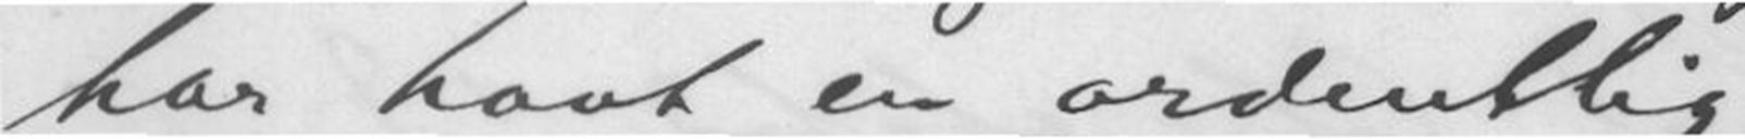har havt en ordentlig
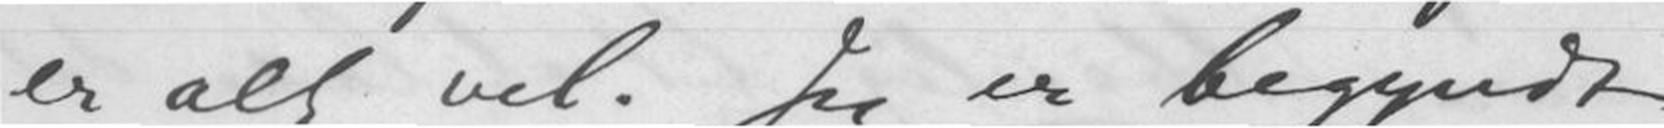er alt vel. Jeg er begyndt
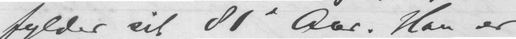fylder sit 81de Aar. Han er
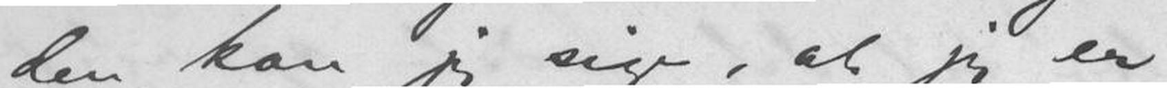den kan jeg sige, at jeg er
